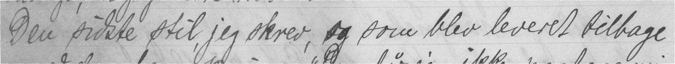Den sidste stil, jeg skrev, og som blev leveret tilbage
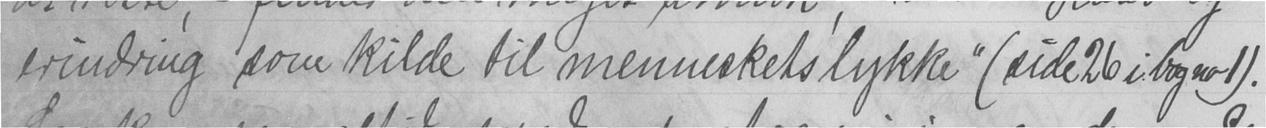erindring som kilde til menneskets lykke" (side 26 i bog no1).
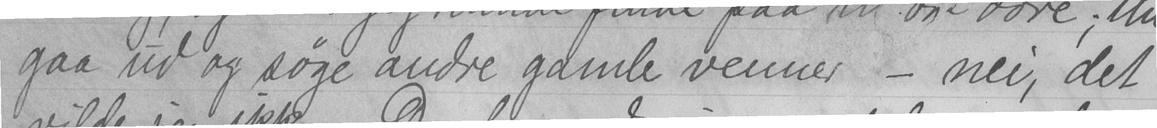gaa ud og søge andre gamle venner - nei, det
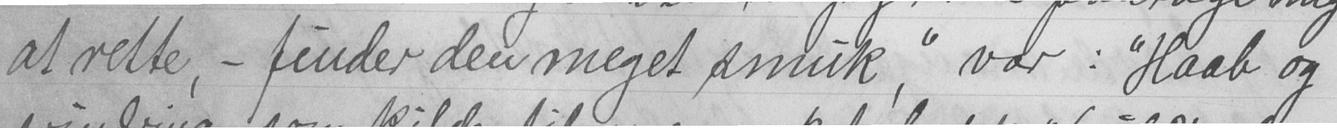at rette, - finder den meget smuk," var: "Haab og
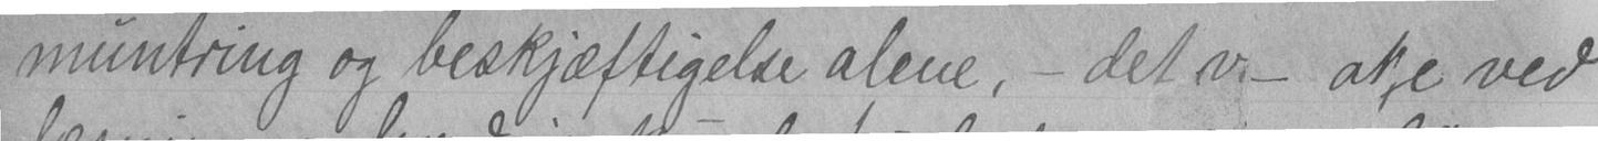muntring og beskjæftigelse alene, - det v- ake ved
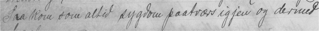Saa kom som altid sygdom paatværs igjen og dermed
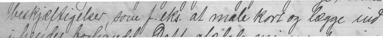beskjæftigelser, som feks. at male kort og lægge ind
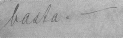basta. -
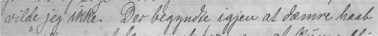vilde jeg ikke. Der begyndte igjen at dæmre haab
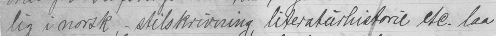lig i norsk, - stilskrivning, literaturhistorie etc. laa
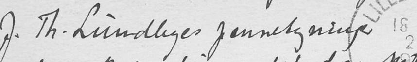J.Th. Lundbyes pennetegninger
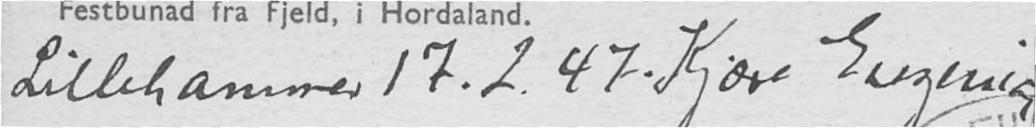Lillehammer 17.2.47. Kjære Eugenia,
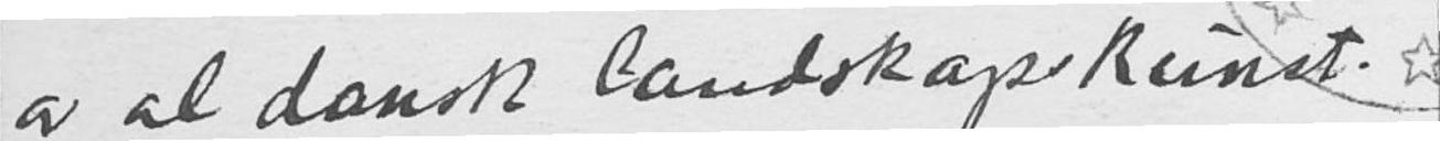av al dansk landskapskunst.
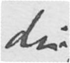din
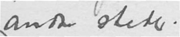andre steder.
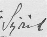Sigrid
